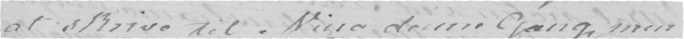at skrive til Nina denne Gang, men
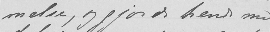melse, og gjorde hende med
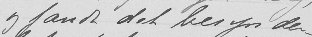og fandt det besynder-
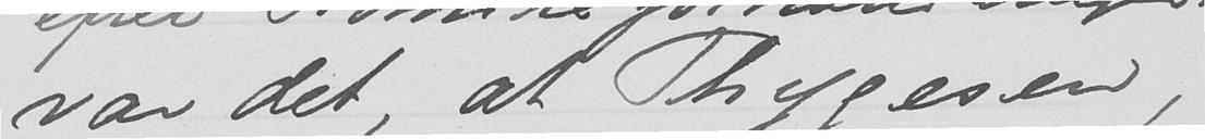var det, at Thygesen,
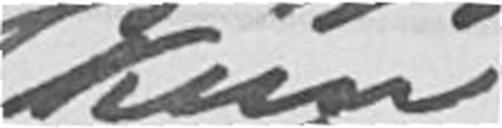kun
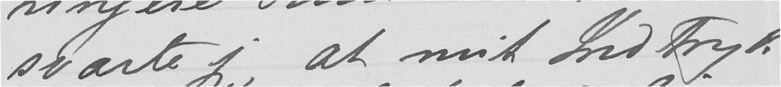svarte jeg at mit Indttryk
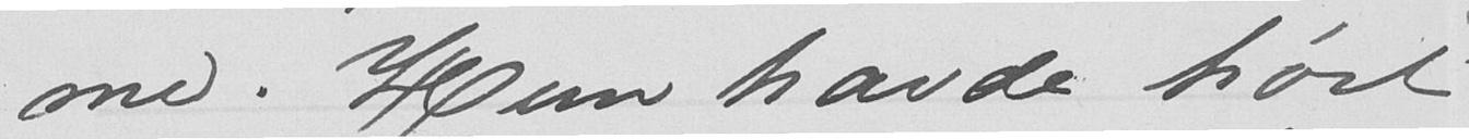med. Hun havde hørt
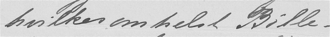hvilkesomhelst Bille-
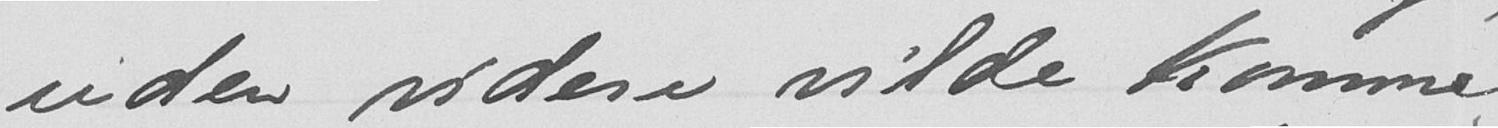uden videre vilde komme
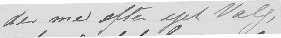der med efter eget Valg,
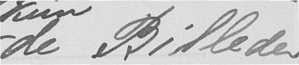de Billeder
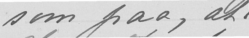som paa, at
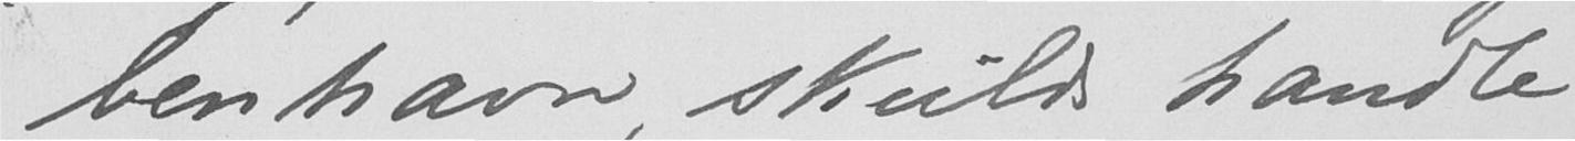venhavn, skulde handle
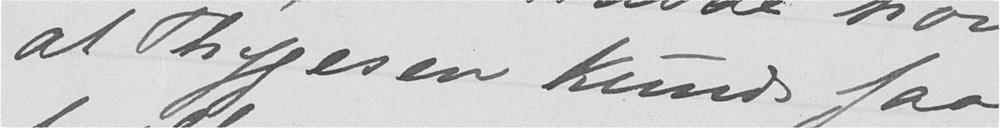at Thygesen kunde faa
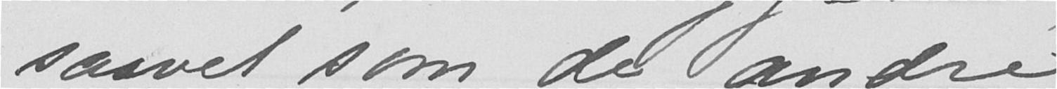saavel som de andre
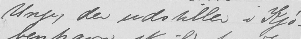Unge, der udstiller i Kjø-
